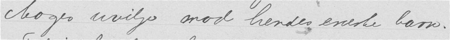Aages uvilje mod hendes eneste barn.
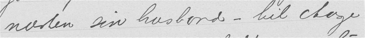næsten sin husbond – til Aaage
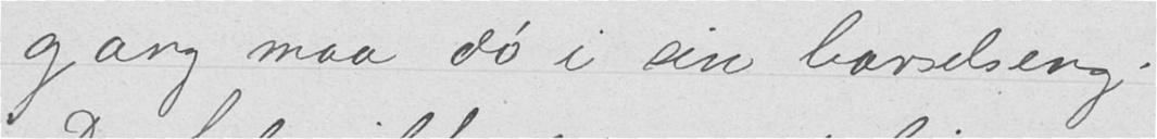gang maa dø i sine barselseng.
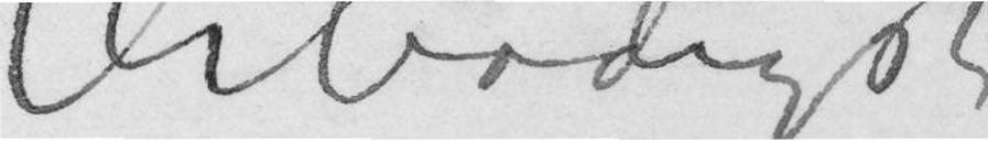Ærbødigst
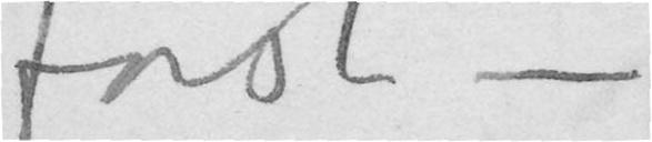først –
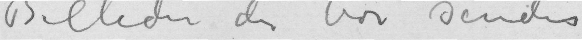Billeder der bør sendes
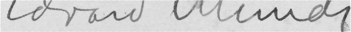Edvard Munch
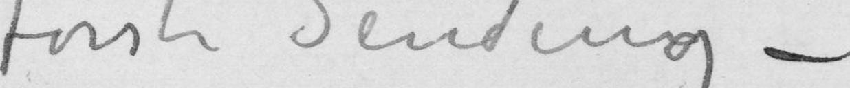første Sending –
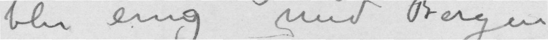blir enig med Bergen
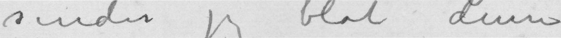sender jeg blot denne
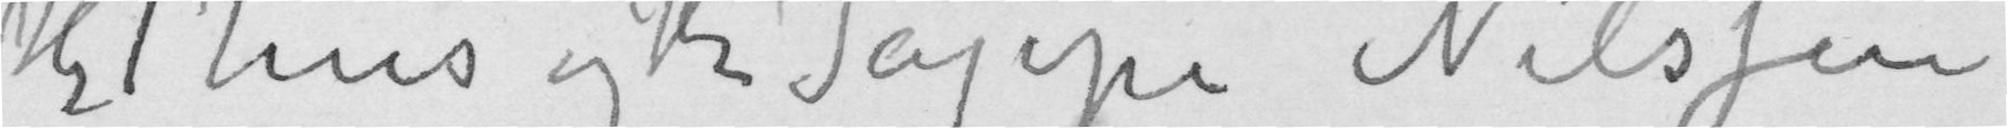Hr Thiis og Hr Jappe Nilssen
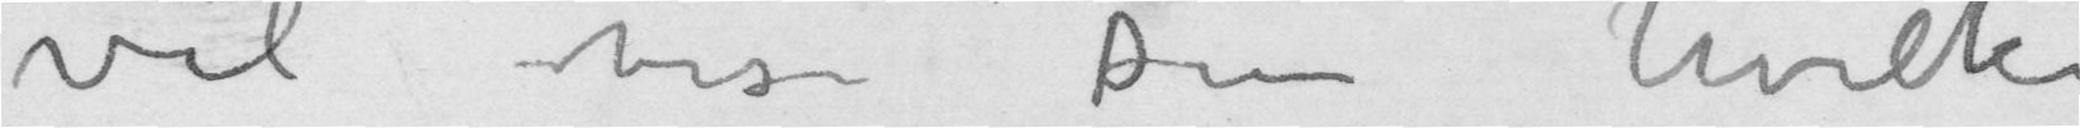vil vise Dem hvilke
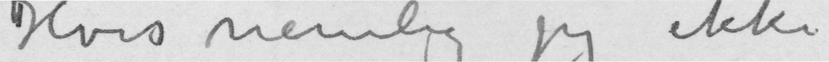Hvis nemlig jeg ikke
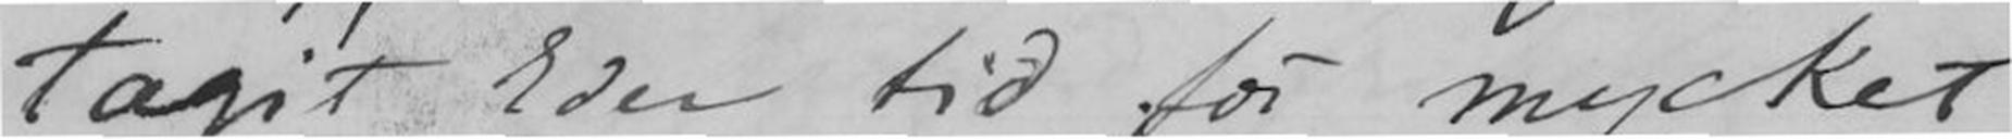tagit Eder tid för mycket
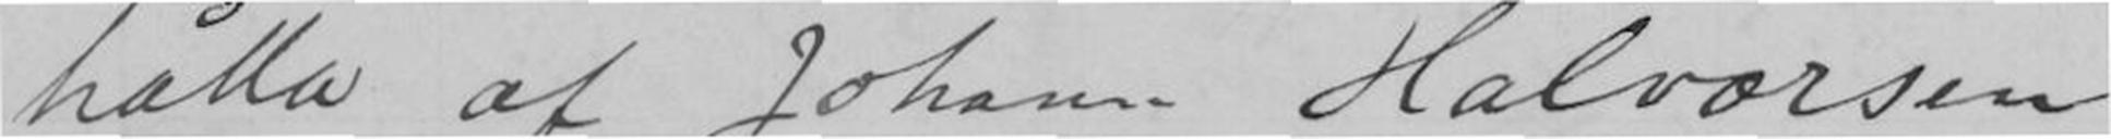hålla af Johann Halvorsen
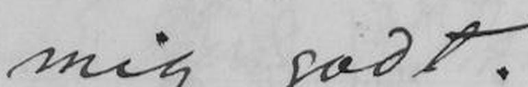mig godt.
